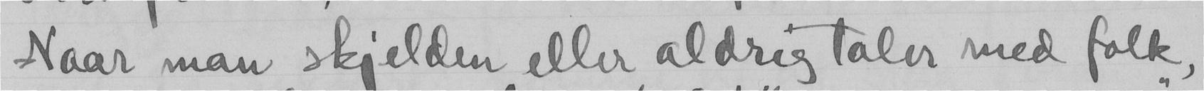Naar man skjelden eller aldrig taler med folk,
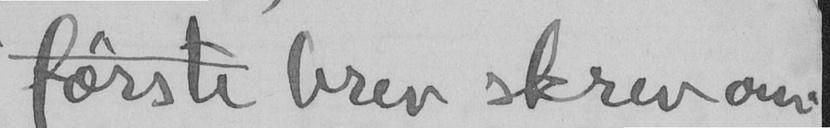første brev skrev om
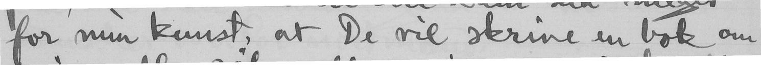for min kunst, at De vel skrive en bok om
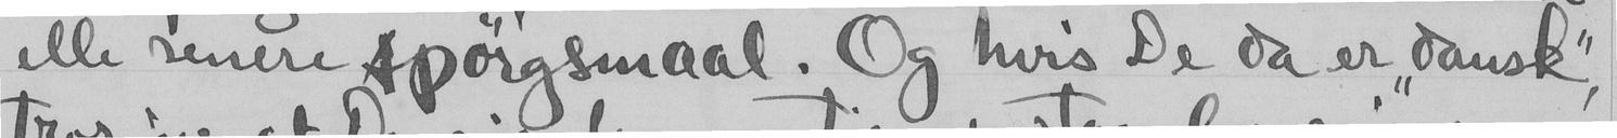elle senere spørgsmaal. Og hvis De da er "dansk",
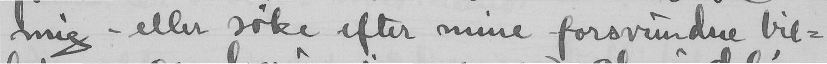mig - eller søke efter mine forsvundne bil-
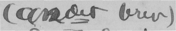(andet brev)
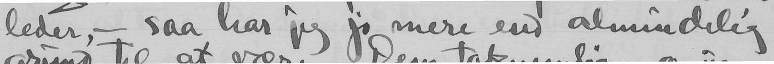leder, - saa har jeg jo mere end almindelig
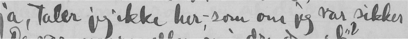ja, taler jeg ikke her som om jeg var sikker
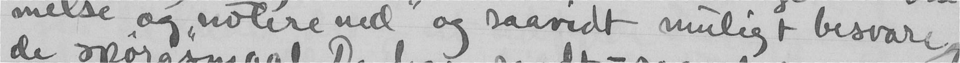melse og "notere ned" og saavidt muligt besvare,
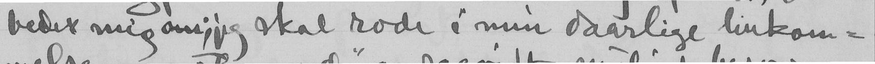beder mig om; jeg skal rode i min daarlige hukom-
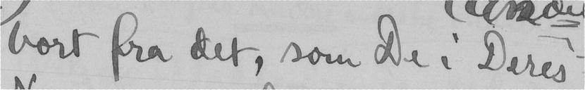bort fra det, som de i Deres
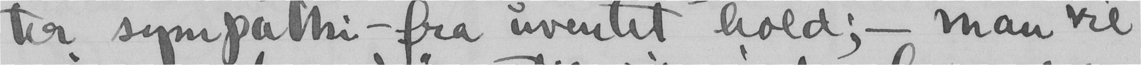ter sympathi - fra uventet hold; - man vil
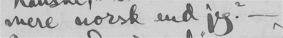mere norsk end jeg? -
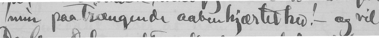min paatrængende aabenkjærtethed! - og vil
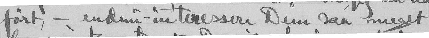ført, - endnu - interessere Dem saa meget
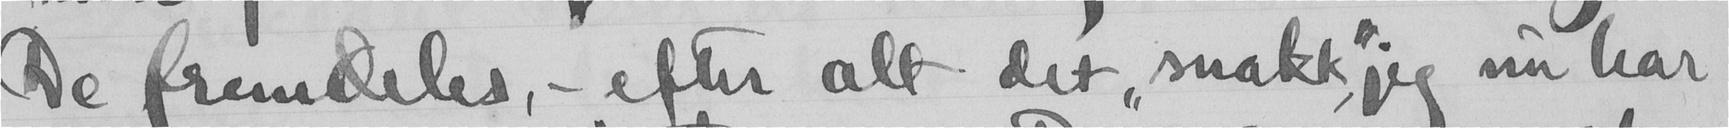De fremdeles, - efter alt det "snakk", jeg nu har
